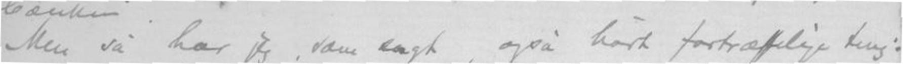Men så har jeg som sagt, også hørt fortræffelige ting.
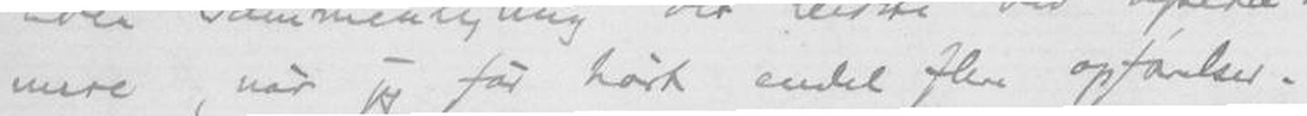mere, når jeg får hørt endel flere opførelser.
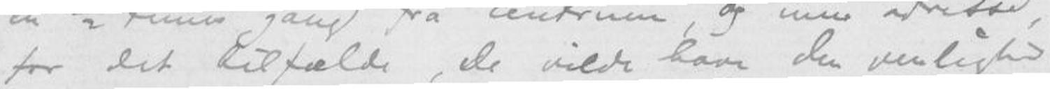for det tilfælde, De vilde have den venlighed
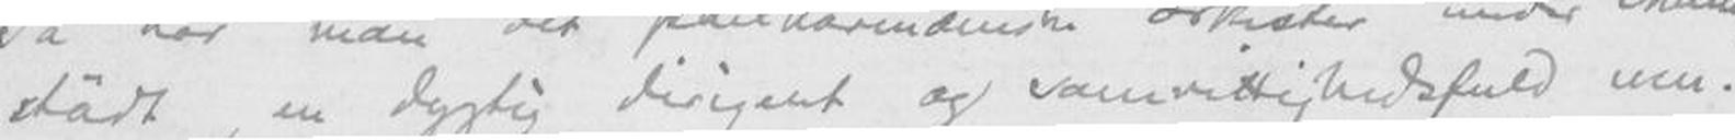städt, en dygtig dirigent og samvittighedsfuld mu-
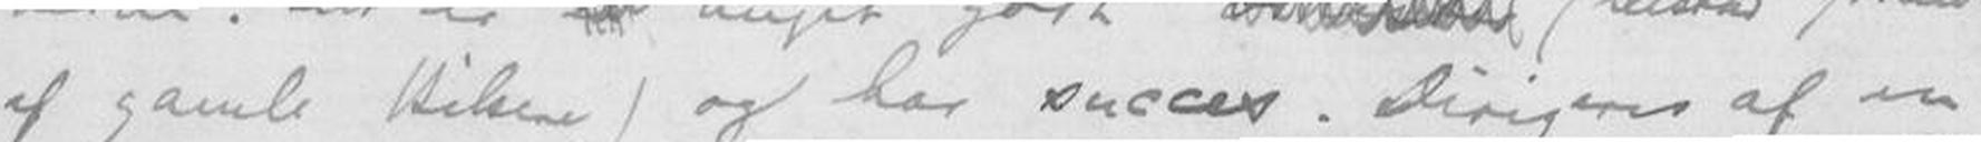af gamle Hilsene) og har succes. Dirigeres af en
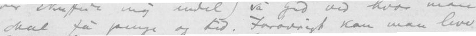skal få penge og tid. Forøvrigt kan man leve
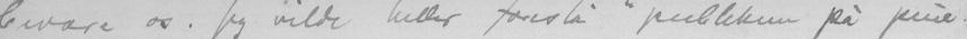bevare os. Jeg vilde heller foreslå "publikum på pine
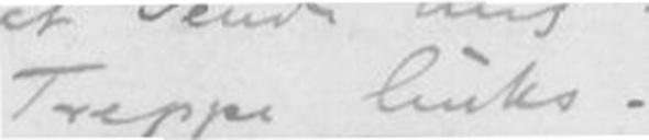Trappe links.
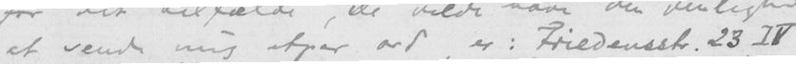at sænde mig etpar ord, er: Friedensstr. 23 IV
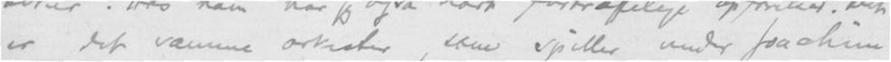er det samme orkester, som spiller under Joachim
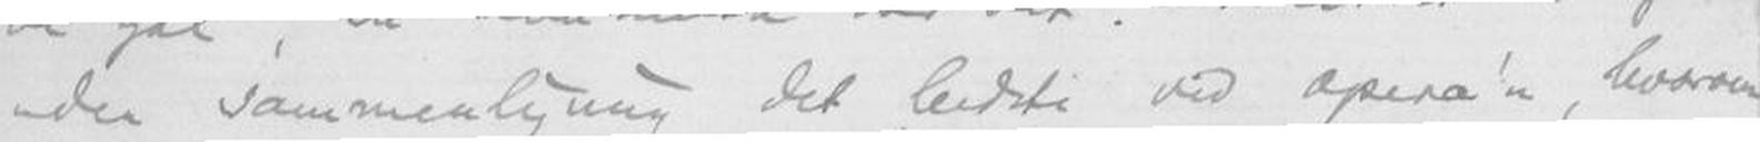uden sammenligning det bedste ved opera'n, hvorom
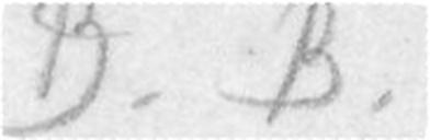B. B.
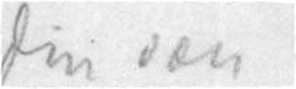Din væn
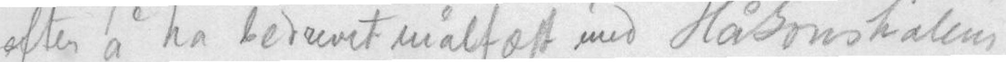efter å ha bedrevet målfæst med Håkonshallen,
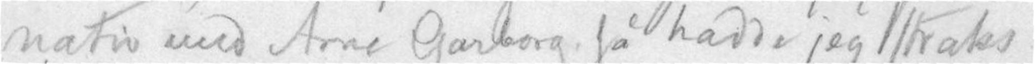nativ med Arne Garborg så hadde jeg straks
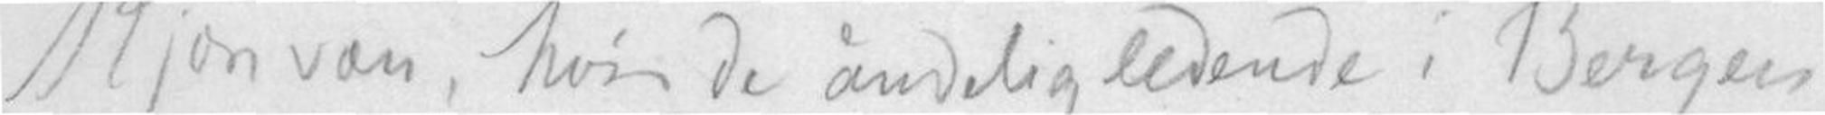Kjære væn, hvis de åndelig ledende i Bergen,
